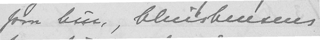forn hus, Christensens
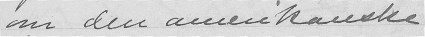om den amerikanske
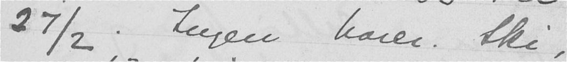27/2. Ingen harer. Ski,
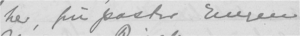her, fru pastor Eugen
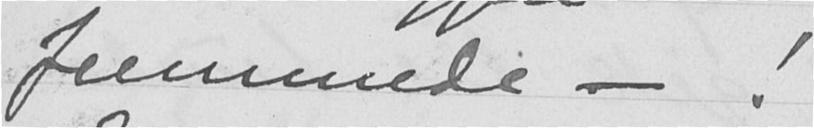fremmede - !
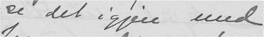se det igjen med
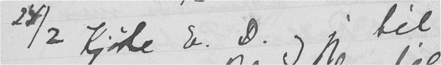24/2 Kjørte E. D. og jeg til
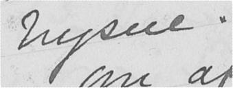Nysne.
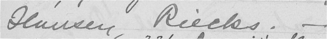Hansen, Riecks. -
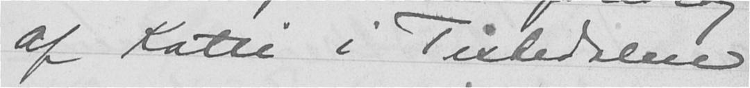af Katti i Tistedalen
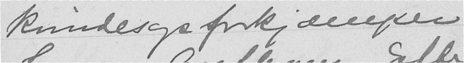kvindesagsforkjæmper
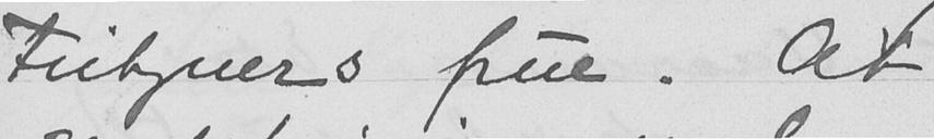Fritzners frue. At
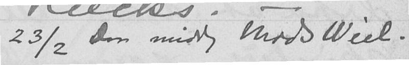23/2 Don middag Mads Wiel.
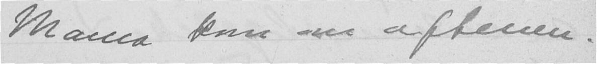Mama kom om aftenen.
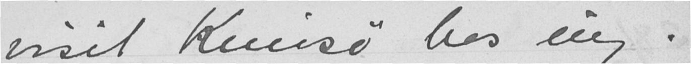visit Knivsø hos ing.
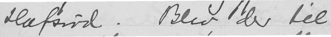Hafsrød. Blev der til
